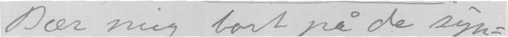Bær mig bort på de syn-
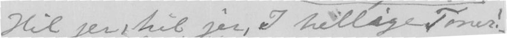Hil jer, hil jer, I hellige Toner!
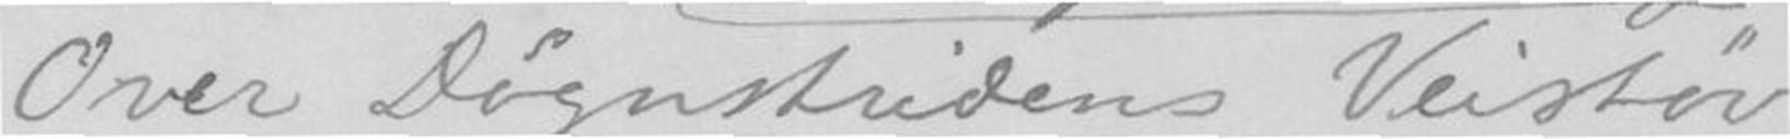Over Døgnstridens Veistøv
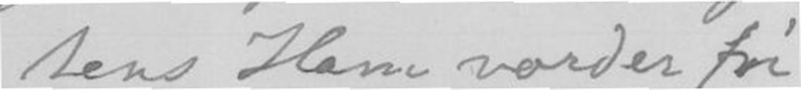tens Ham vorder fri
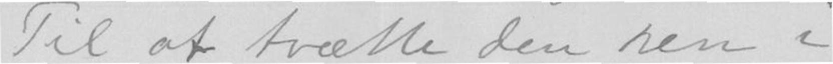Til at tvætte den ren i
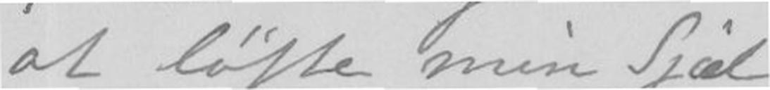at løfte min Sjæl
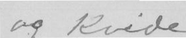og Kvide!
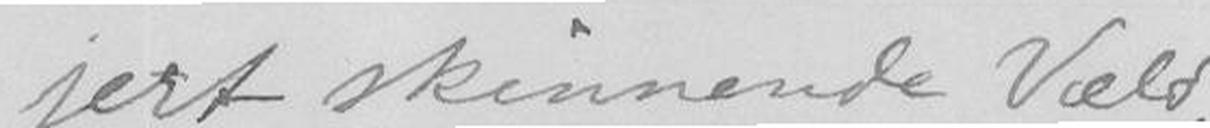jert skinnende Væld,
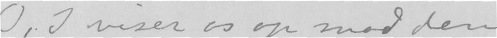O, I viser os op mod den
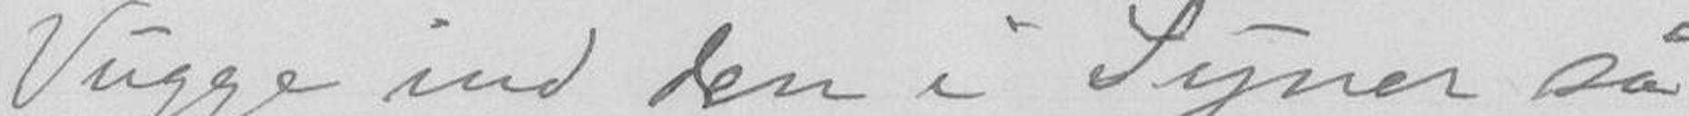Vugge ind den i Syner så
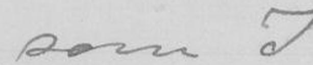som I,
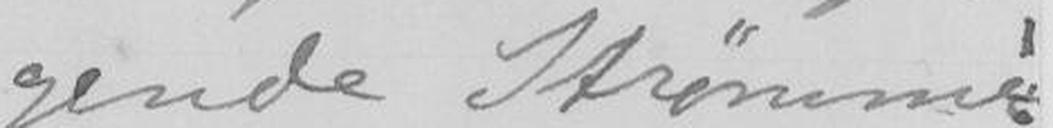gende Strømme
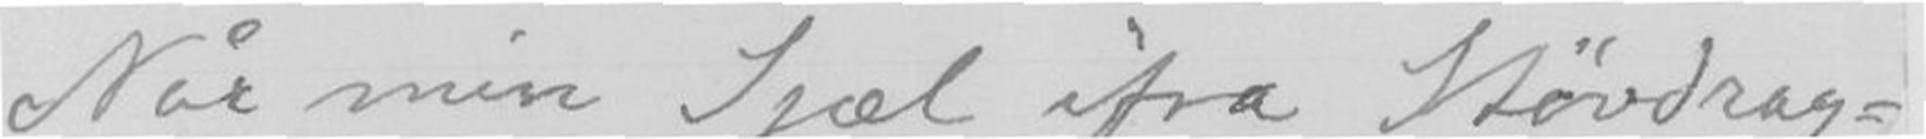Når min Sjæl ifra Støvdrag-
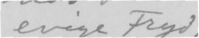evige Fryd,
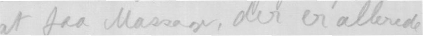at faa massage, der er allerede
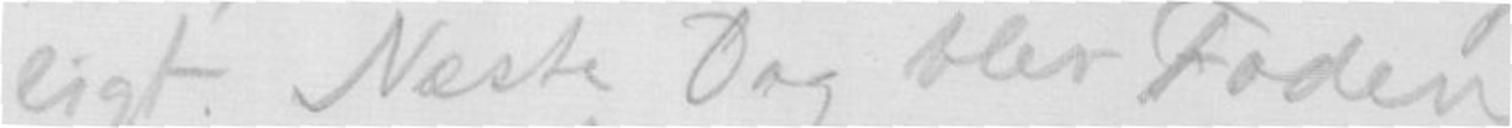ligt. Næste Dag blir Foden
